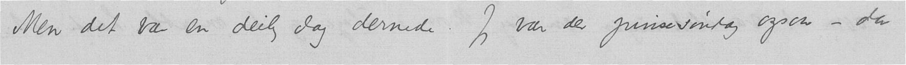Men det var en deilig dag dernede. Jeg var der pinsesøndag ogsaa – da
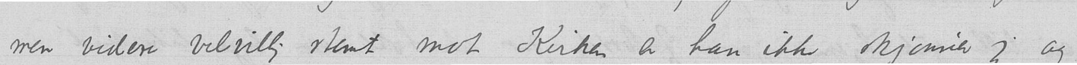men videre velvillig stemt mot Kirken er han ikke skjønner jeg, og
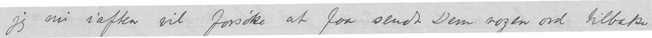jeg nu iaften vil forsøke at faa sendt Dem nogen ord tilbake
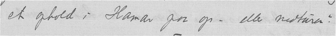et ophold i Hamar paa op – eller nedturen?
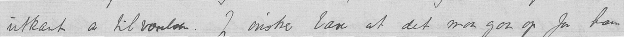utkant av tilværelsen. Jeg ønsker bare at det maa gaa op for ham
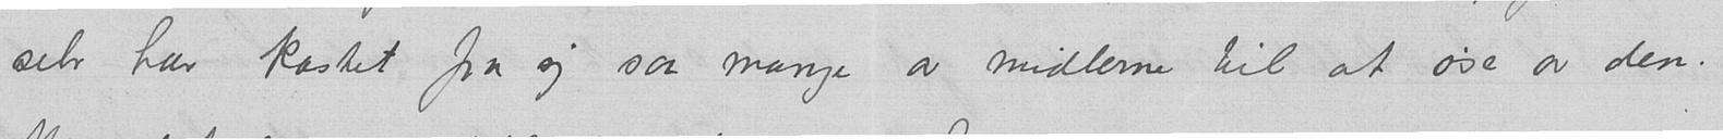selv har kastet fra sig saa mange av midlerne til at øse av den.
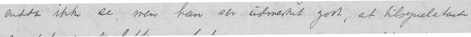endda ikke se, men han ser udmerket godt, at tilsynelatende
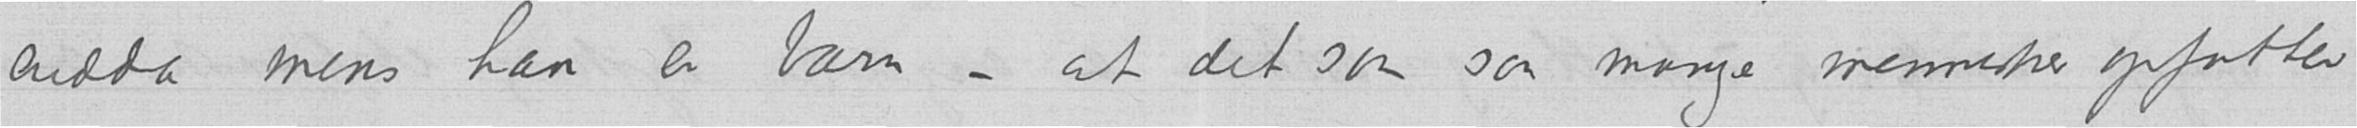endda mens han er barn – at det som saa mange mennesker opfatter
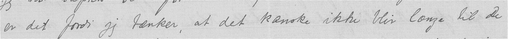er det fordi jeg tænker, at det kanske ikke blir længe til de
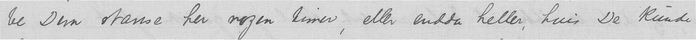be Dem stanse her nogen timer, eller endda heller, hvis De kunde
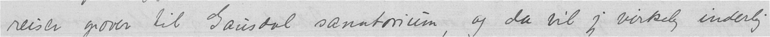reiser opover til Gausdal sanatorium, og da vil jeg virkelig inderlig
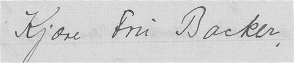Kjære Fru Backer,
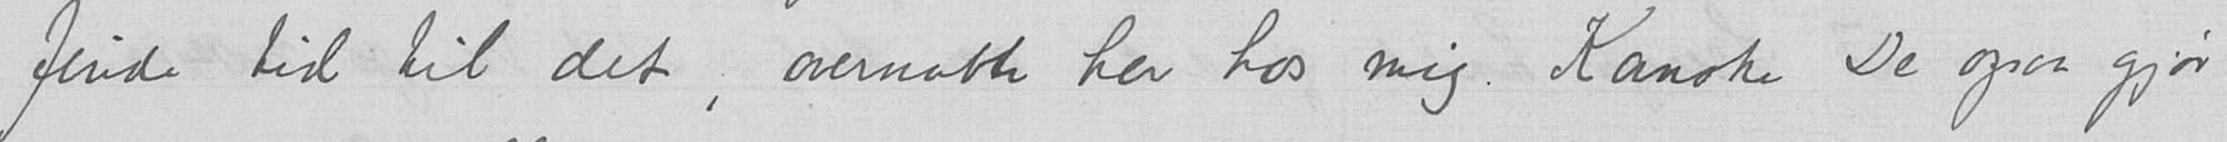finde tid til det; overnatte her hos mig. Kanske De ogsaa gjør
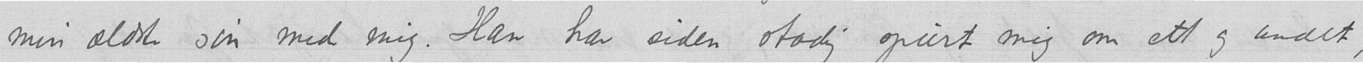min ældste søn med mig. Han har siden stadig spurt mig om ett og andet,
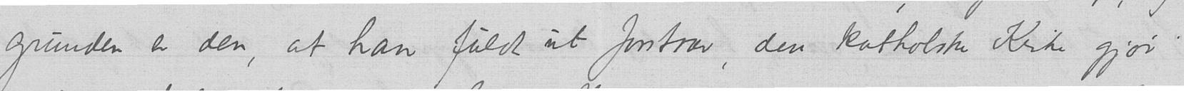grunden er den, at han fuldt ut forstaar, den katholske Kirke gjør
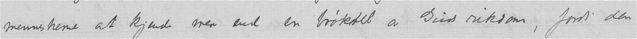menneskerne at kjende mere end en brøkdel av Guds rikdom, fordi den
